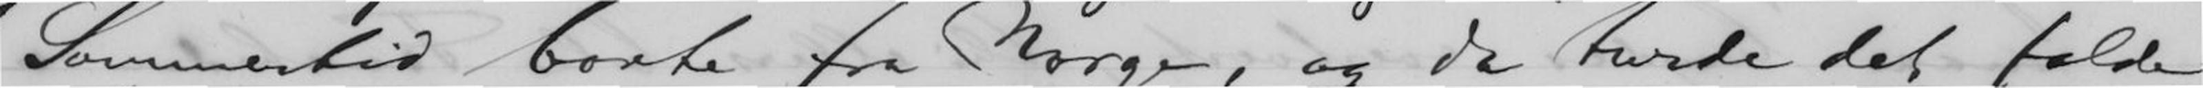Sommertid borte fra Norge, og da turde det falde
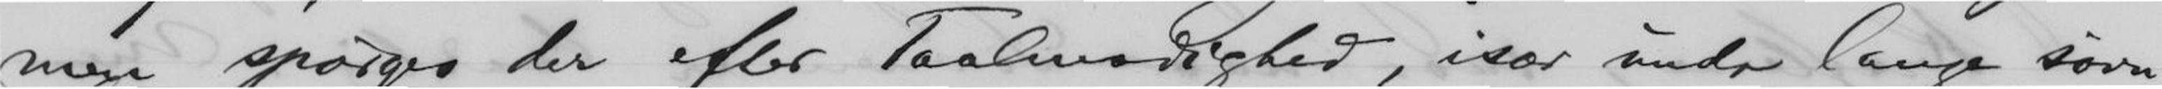men spørges der efter Taalmodighed, især under lange søvn
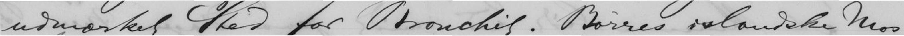udmærket Sted for Bronchit. Børres islandske Mos
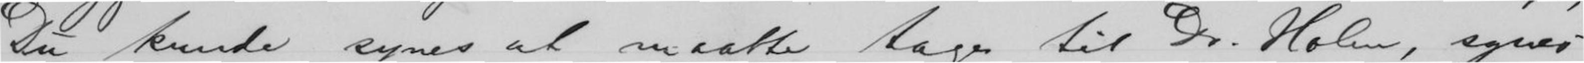Du kunde synes at maatte tage til Dr. Holm, synes
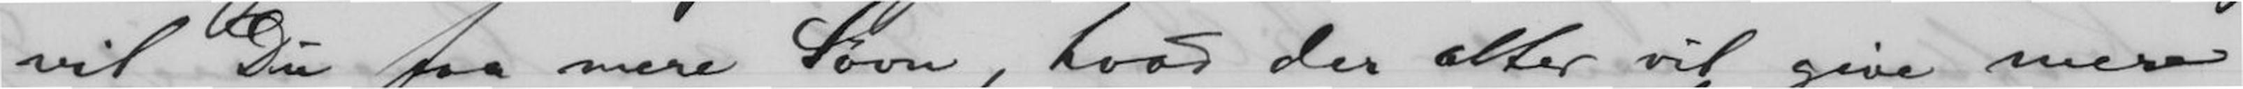vil Du faa mere Søvn, hvad der atter vil give mere
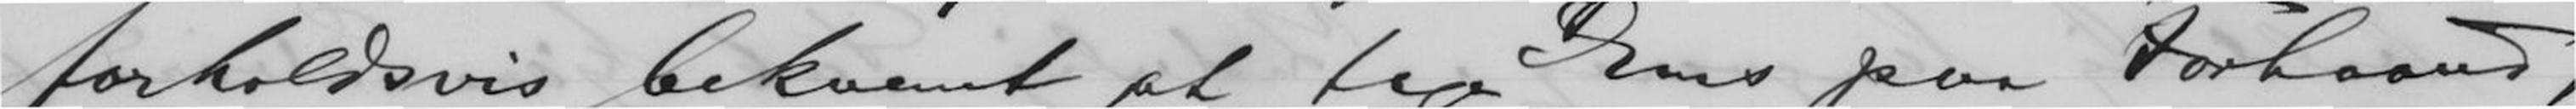forholdsvis bekvemt at tage Ems paa Forhaand,
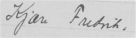Kjære Fredrik,
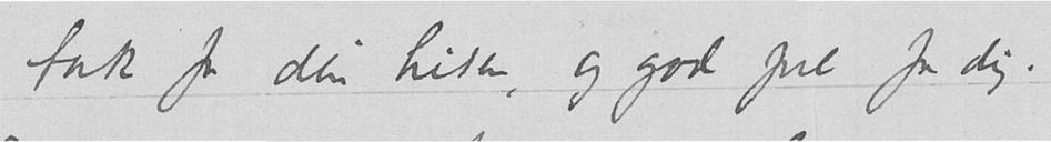tak for din hilsen, og god jul for dig.
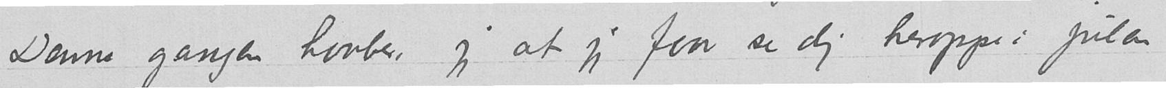Denne gangen haaber jeg at jeg faar se dig heroppe i julen
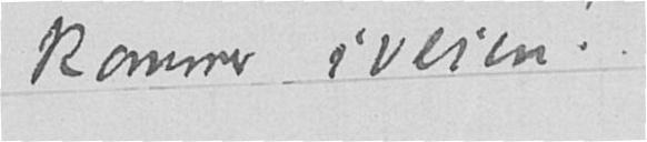kommer iveien!
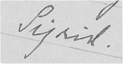Sigrid.
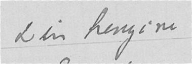din hengivne
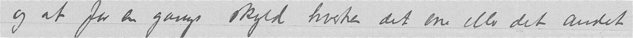og at for en gangs skyld hverken det ene eller det andet
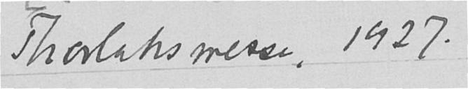Thorlaksmesse, 1927.
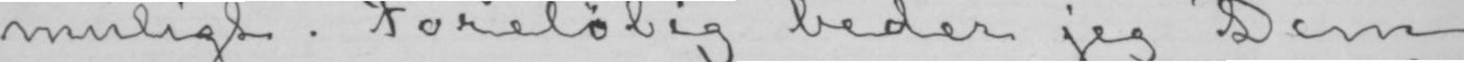muligt. Foreløbig beder jeg Dem
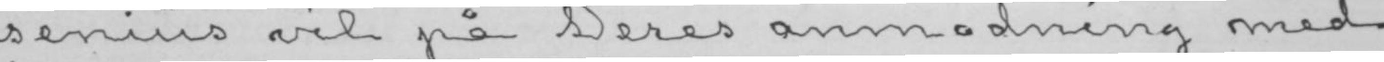senius vil på Deres anmodning med
